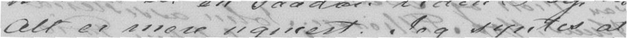Alt er mere ugenert. Jeg syntes at
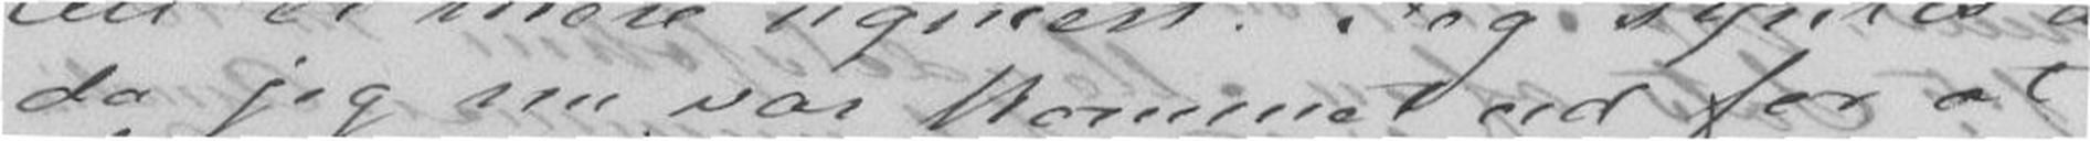da jeg nu var kommet ud for at
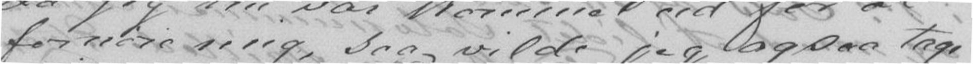fornøie mig, saa vilde jeg ogsaa tage
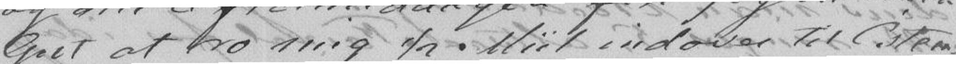Gut at ro mig ½ Miil indover til Østen-
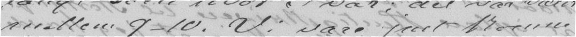mellem 9-10. Vi vare just komne
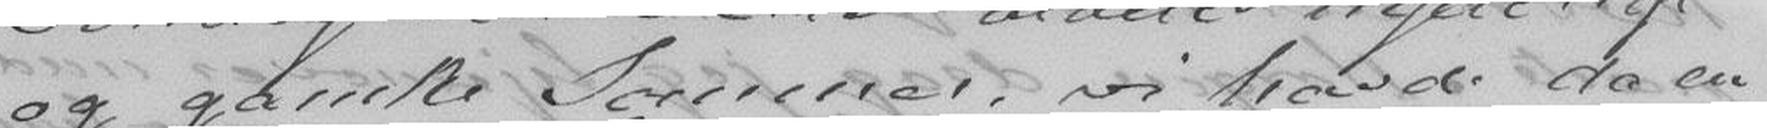og ganske Sommer, vi havde da en
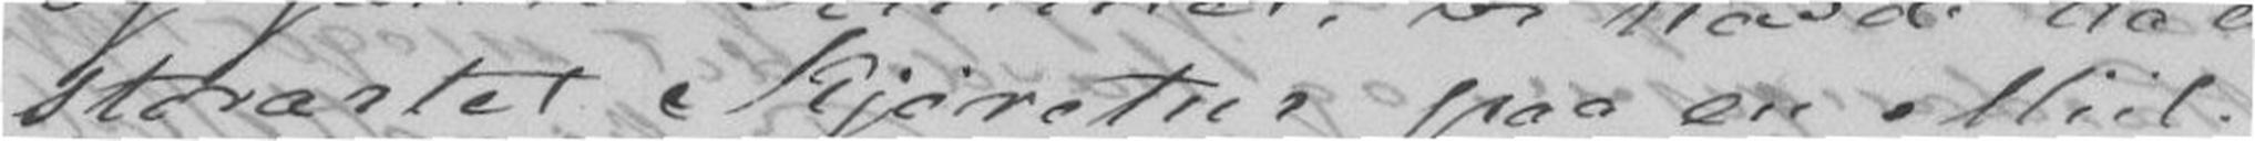storartet Kjøretur paa en Miil.
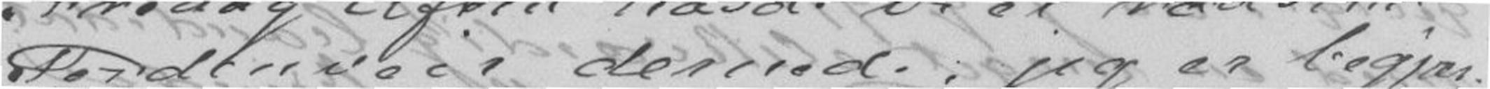Tordenveir dernede, jeg er begjær-
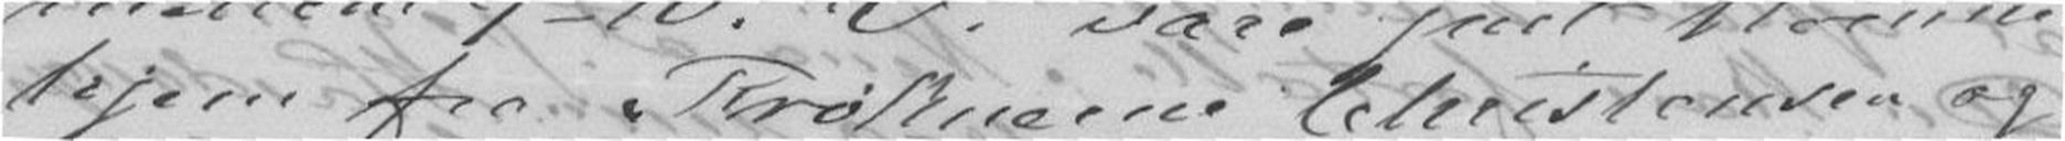hjem fra Frøknerne Christensen og
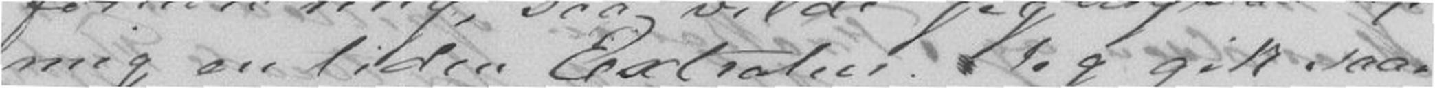mig en liden Extratur. Jeg gik saa-
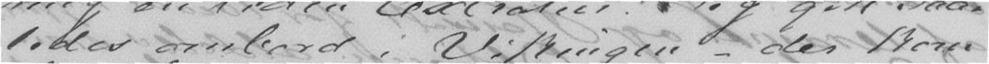ledes ombord i Vikingen - der kom
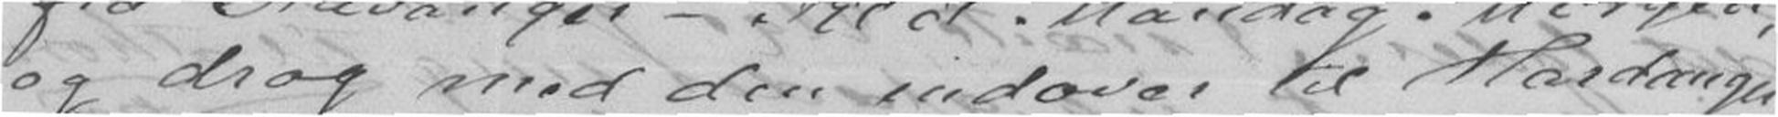og drog med den indover til Hardanger,
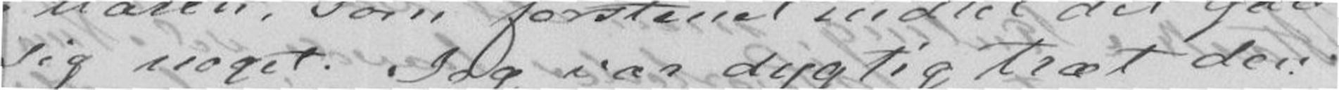sig noget. Jeg var dygtig træt den
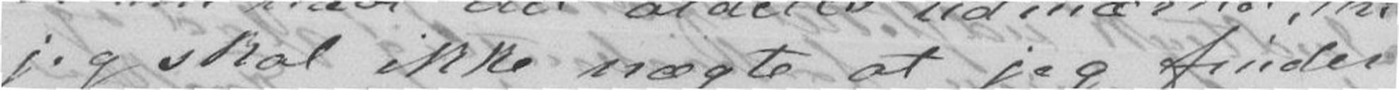jeg skal ikke nægte at jeg finder
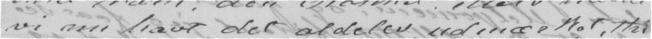vi nu havt det aldeles udmærket, thi
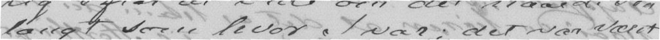langt som hvor I var; det var været
